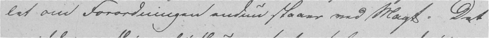let om Forordningen endnu staaer ved Magt. Det
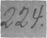224.
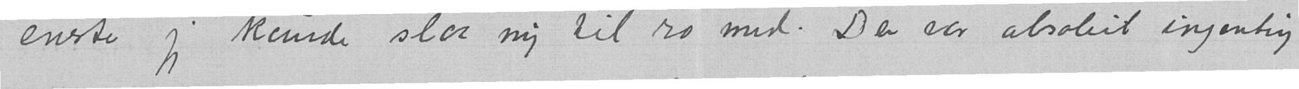eneste jeg kunde slaa mig til ro med. Der var absolut ingenting
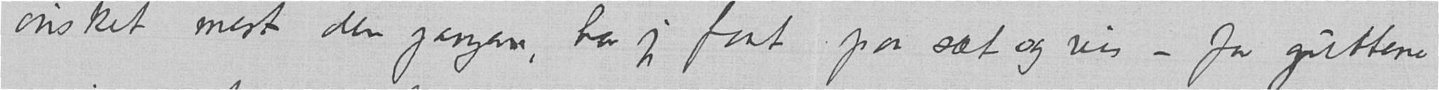ønsket mest den gangen, har jeg faat paa sæt og vis – for guttene
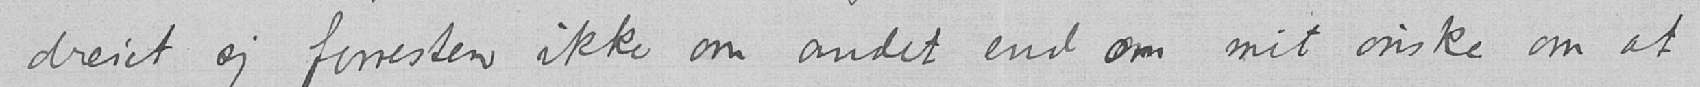dreiet sig forresten ikke om andet end om mit ønske om at
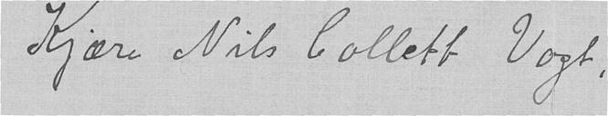Kjære Nils Collett Vogt,
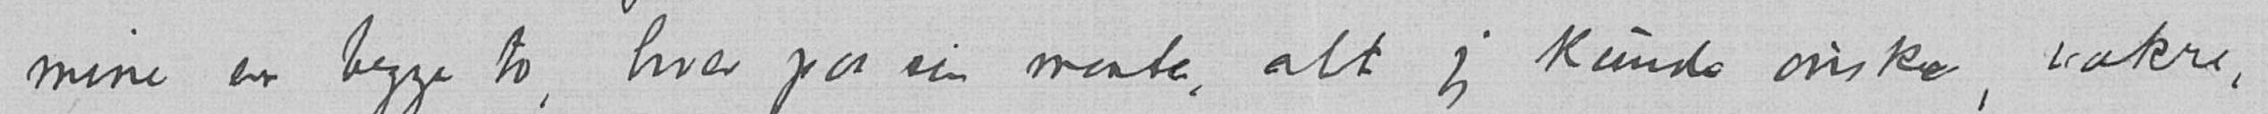mine er begge to, hver paa sin maate, alt jeg kunde ønske, vakre,
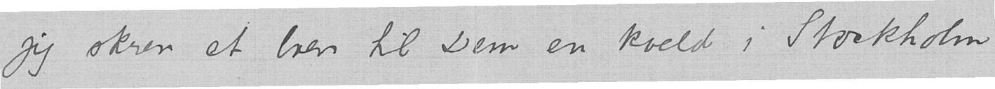jeg skrev et brev til Dem en kveld i Stockholm
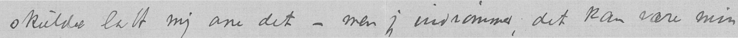skulde latt mig ane det - men jeg indrømmer; det kan være min
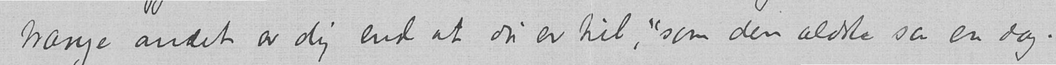trænge andet av dig end at du er til," som den ældste sa en dag.
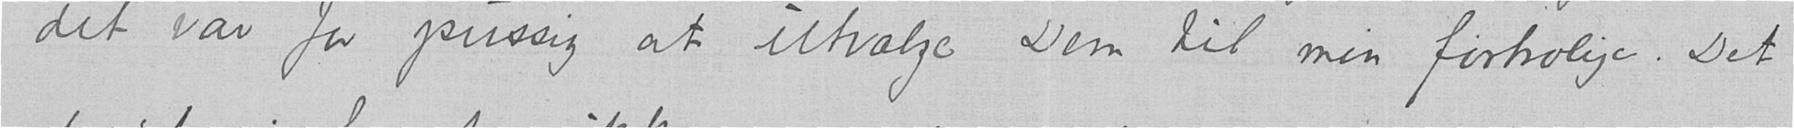det var for pussig at utvælge Dem til min fortrolige. Det
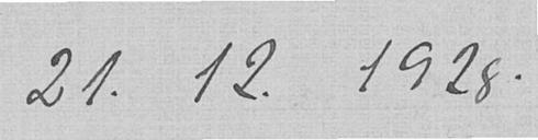21.12.1928.
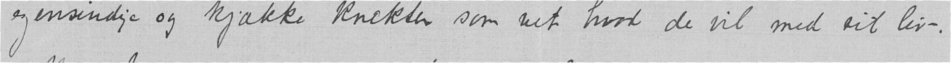egensindige og kjække knekter som vet hvad de vil med sit liv-.
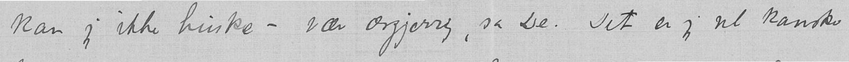kan jeg ikke huske – vær ærgjerrig, sa De. Det er jeg vel kanske
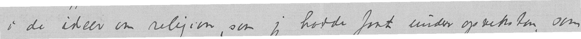i de ideer om religion, som jeg hadde faat under opveksten, som
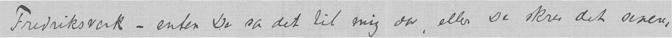Fredriksverk - enten De sa det til mig da, eller De skrev det senere,
